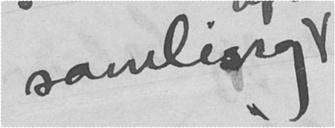samling
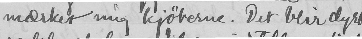mærket mig kjøberne. Det blir dyrt
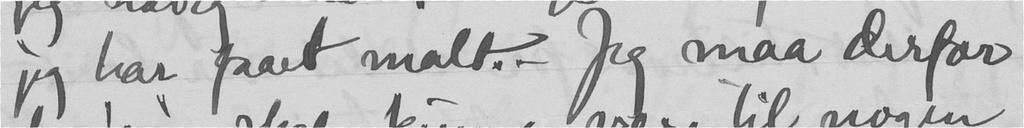jeg har faaet malt.- Jeg maa derfor
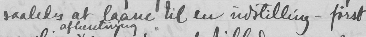saaledes at laane til en udstilling - først
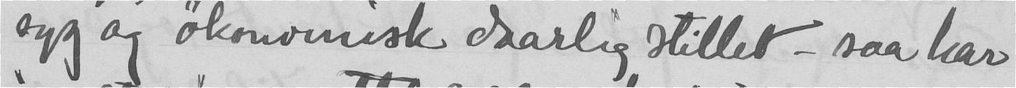syg og økonomisk daarlig stillet, - saa har
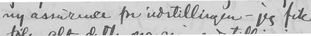ny assurance for udstillingen - jeg fik
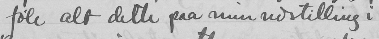føle alt dette paa min udstilling i
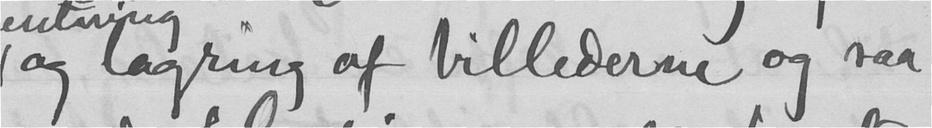og lagring af billederne og saa
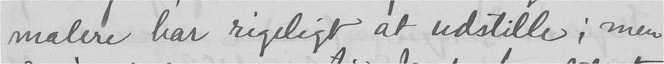malere har rigeligt at udstille; men
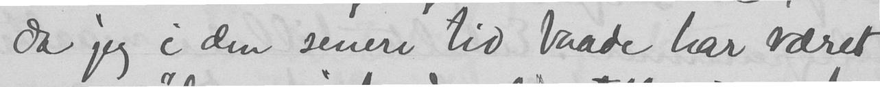da jeg i den senere tid baade har været
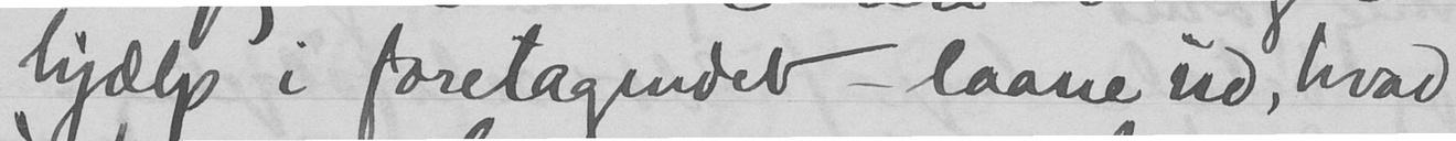hjælp i foretagendet laane ud, hvad
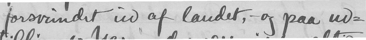forsvundet ud af landet, - og paa ud-
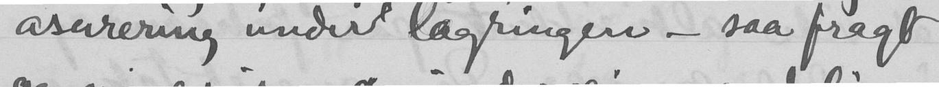asurering under lagringen, - saa fragt
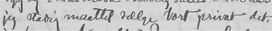jeg stadig maattet sælge bort privat det,
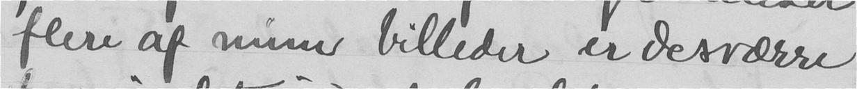flere af mine billeder er desværre
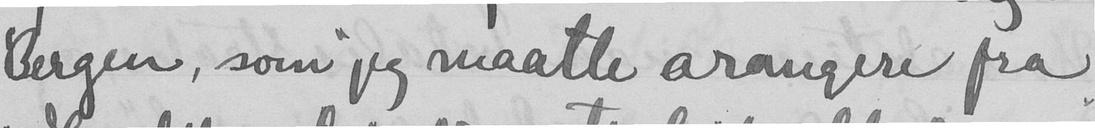Bergen, som jeg maatte arangere fra
